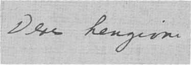Deres hengivne
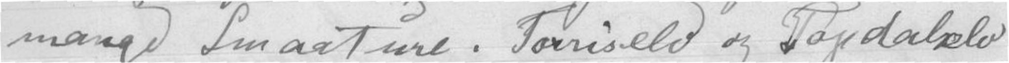mange Smaature. Torriselv og Topdalselv
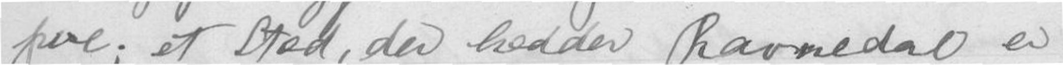pere; et Sted, der hedder Ravnedal er
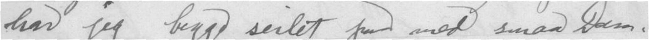hr jeg begge seilet paa med smaa Dam-
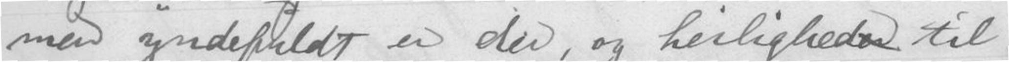men yndefuldt er der, og herligheden til
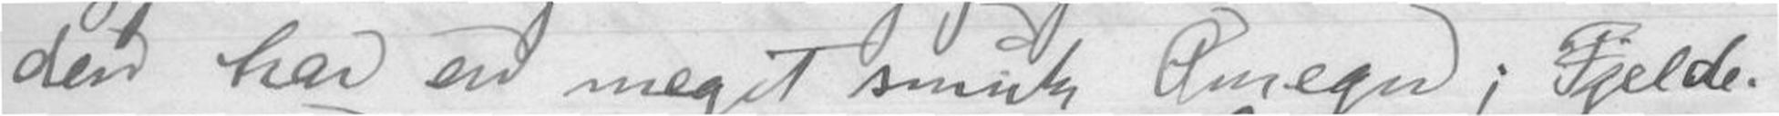den har en meget smuk Omegn; Fjelde-
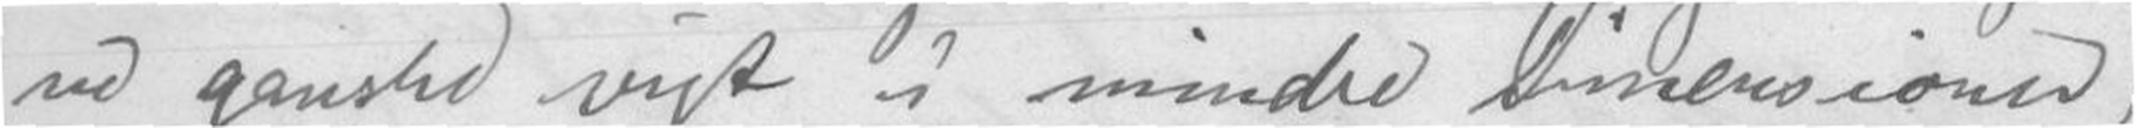ne ganske vist i mindre Dimensioner,
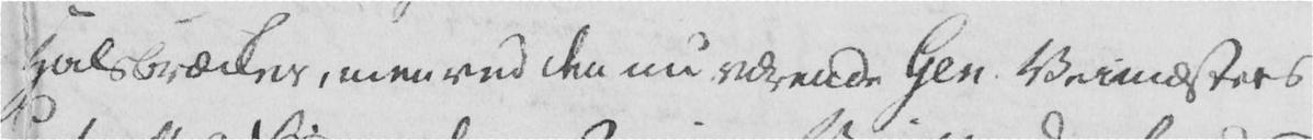Halsbræcken, men ved den nu værende Gen. Veimæsters
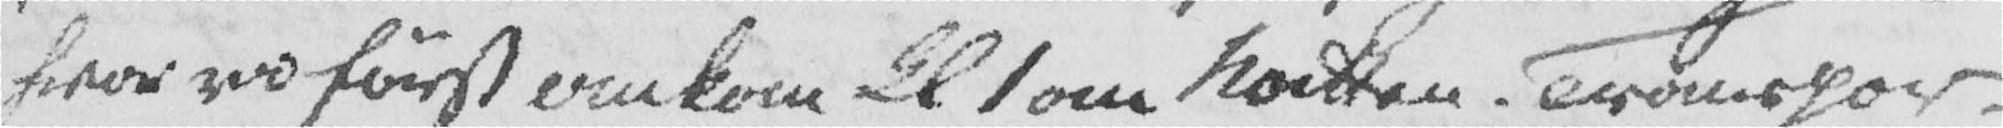hvor vi først ankom kl 1 om Natten. Transpor-
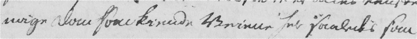mige Dom som kiende Veiene her saaledes som
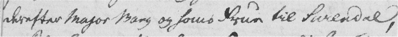derefter Major Bang og hans Frue til Surendal,
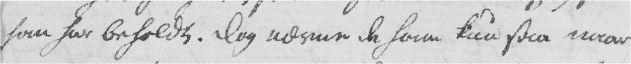han har beholdt. Dog nævne de ham kun saa naar
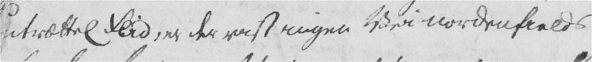utrættel Flid, er der vist ingen Vei nordenfields
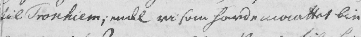til Tronhiem; endl vi som havde maattet bie
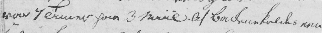var 7 Timer paa 3 Miil. Af Backene kaldes een
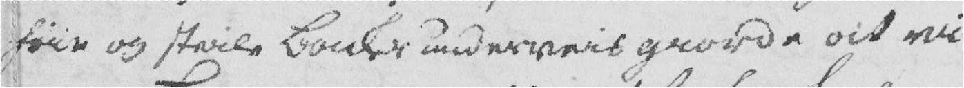høie og steile Backer underveis giorde at vi
